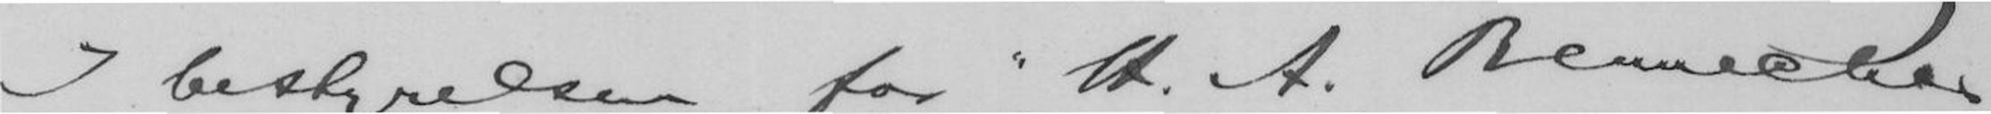I bestyrelsen for "H. A. Benneches
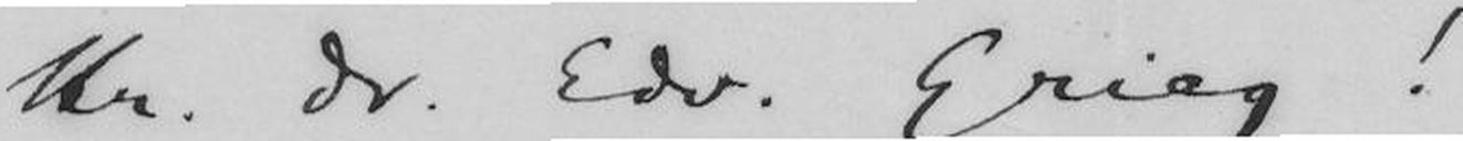Hr. Dr. Edv. Grieg!
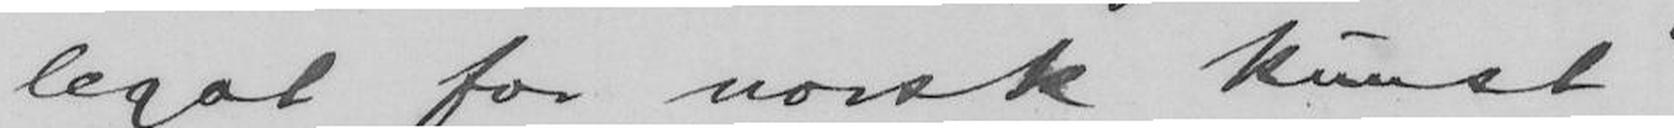legat for norsk kunst"
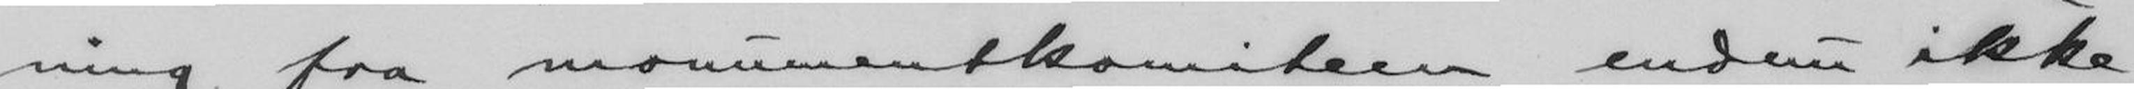ning fra monumentkomiteen endnu ikke
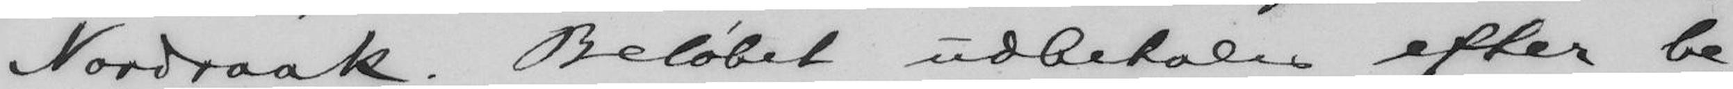Nordraak. Beløbet udbetales efter be-
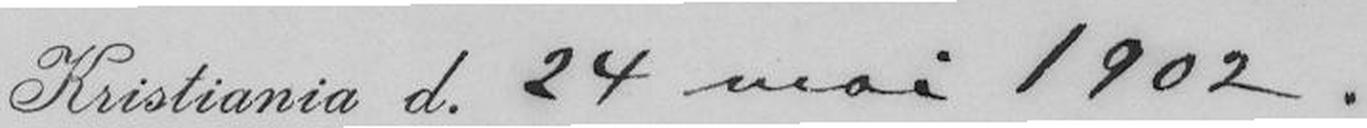Kristiania d. 24 mai 1902.
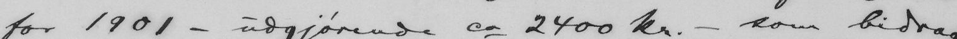for 1901 - udgjørende ca 2400 kr. - som bidrag
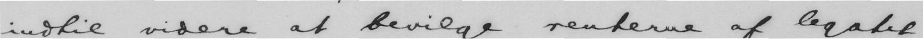indtil videre at bevilge renterne af legatet
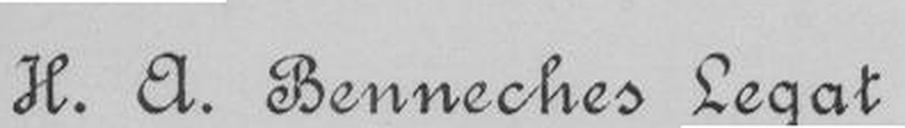H. A. Benneches Legat
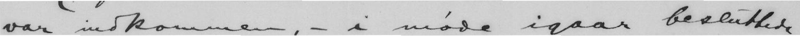var indkommen, - i møde igaar besluttede
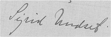Sigrid Undset.
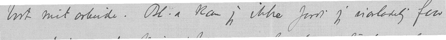bort mit arbeide. Bl.a kan jeg ikke fordi jeg uavladelig faar
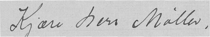Kjære Herr Møller,
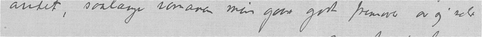andet, saalænge romanen min gaar godt fremover av sig selv
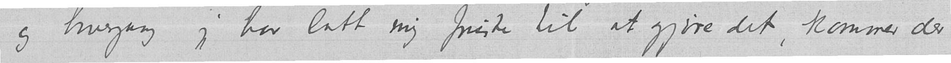og hvergang jeg har latt mig friste til at gjøre det, kommer der
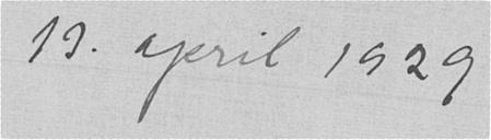13. april 1929
